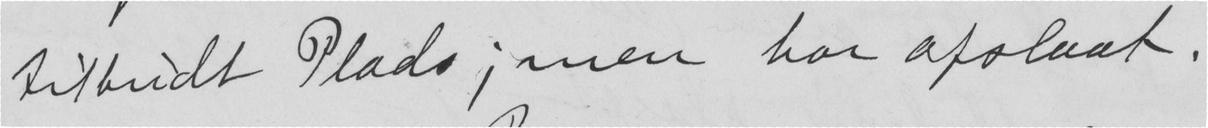tilbudt Plads; men har afslaat.
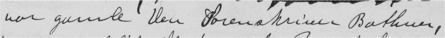vor gamle Ven Sorenskriver Bothner,
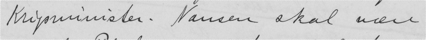Krigsminister. Nansen skal være
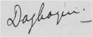Dagbogen.
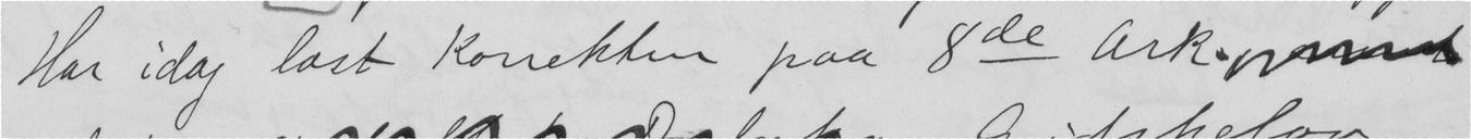Har idag læst Korrektur paa 8de Ark
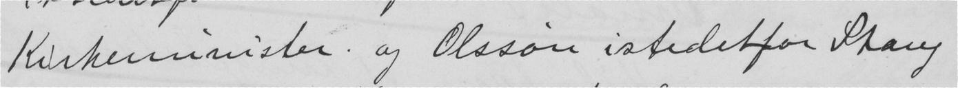Kirkemnister, og Olsson istedetfor Stang
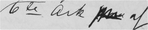6te Ark  at
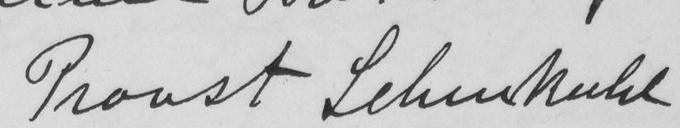Proust Lehmkuhl
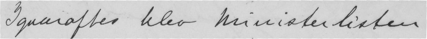Igaaraftes blev ministerlisten
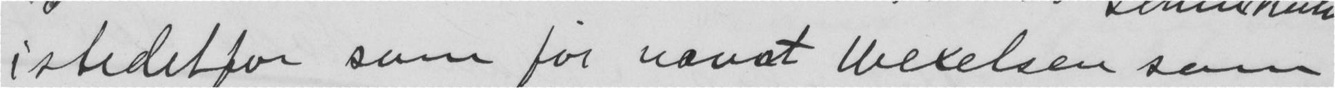istedetfor som før nævnt Wexelsen som
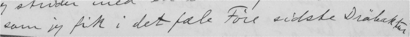som jeg fik i det fæle Føre sidste Drøbaktur.
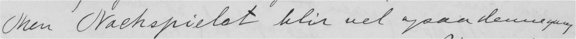Men Nachspielet blir vel ogsaa dennegang
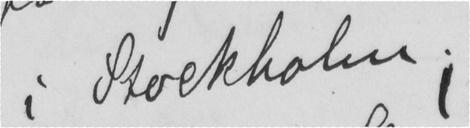i Stockholm;
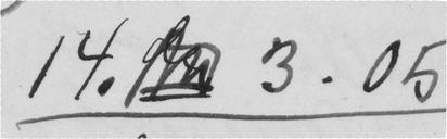14. 3.05
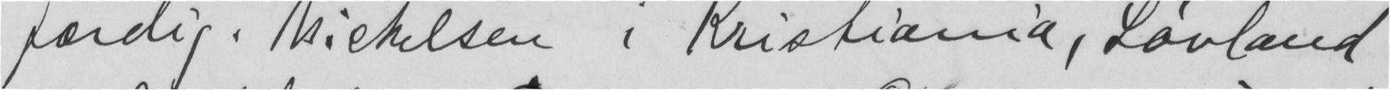færdig. Mickelsen i Kristiania, Løvland
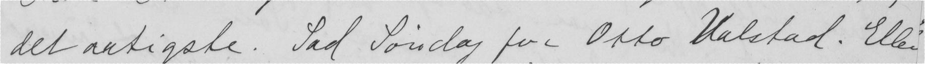det artigste. Sad Søndag for Otto Valstad. Eller
